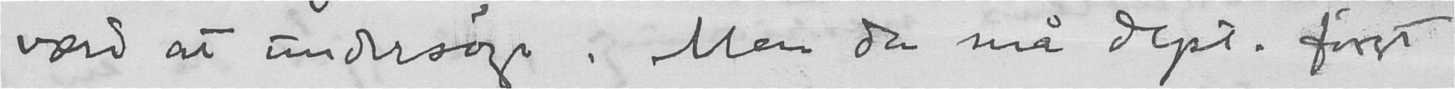værd at undersøge. Men da må dept. først
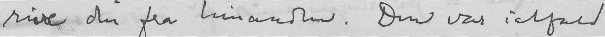rive den fra hinanden. Den var ialfald
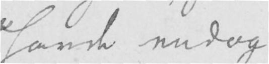havde endog
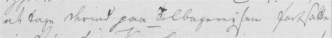at tage derind paa Tilbagereysen fortsatte
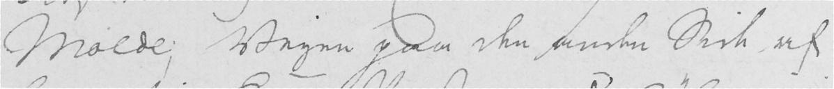Molde; Veyen paa den anden side af
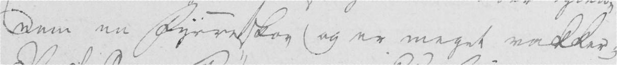nem en Fyrreskov og er meget vakker;
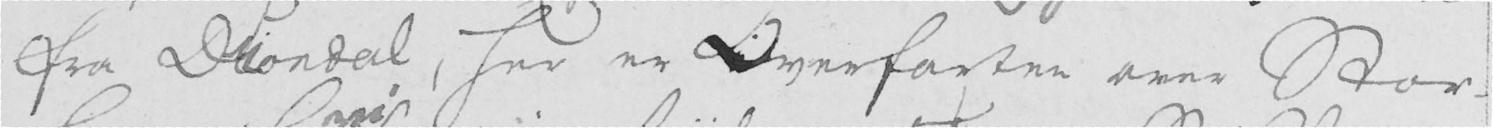fra Diondal, her er Overfarten over Stor-
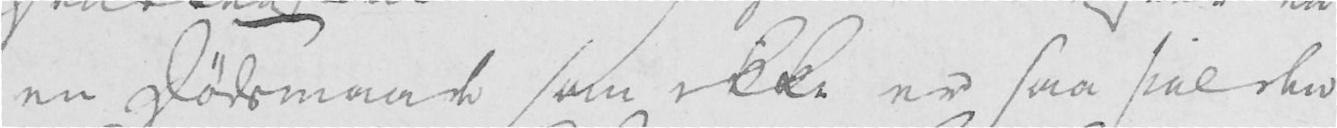en Dødsmaade som ikke er saa sielden
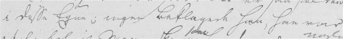i disse Egne; ingen beklagede ham, han var
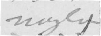nogle
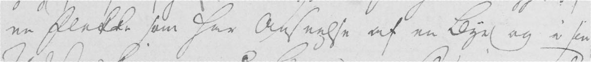en Flekke som har Anseelse af en Bye og i sin
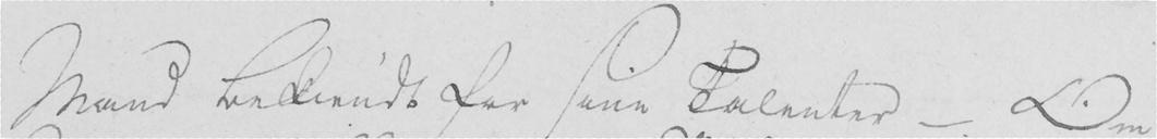Mand bekiendt for sine Talenter - Om
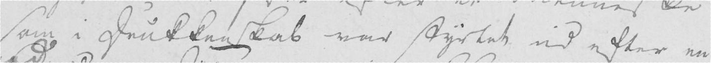som i Drukkenskab var styrtet ud efter en
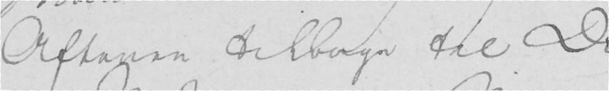Aftenen tilbage til D
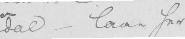dal - laae her
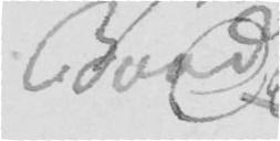Baad
